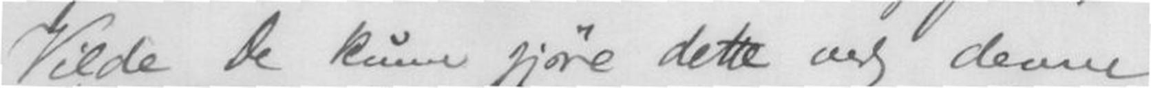Vilde De kunne gjøre dette ved denne
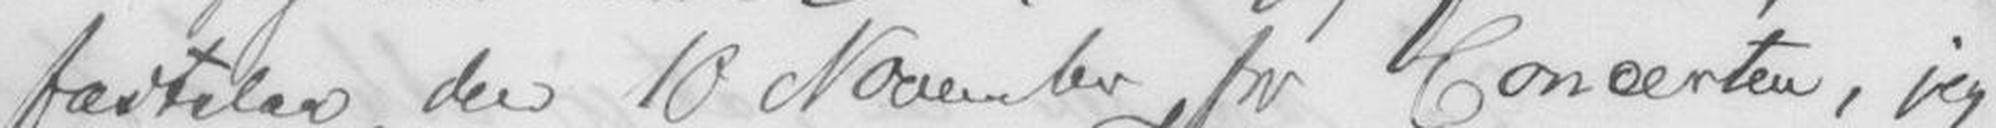den 10 November for Concerten, jeg
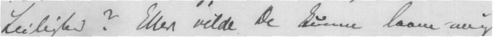Leilighed? Eller vilde De kunne laane mig
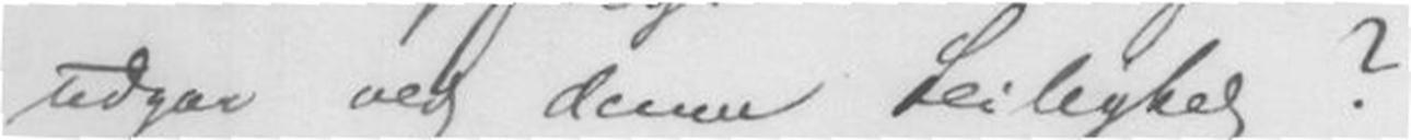udgaa ved denne Leilighed ?
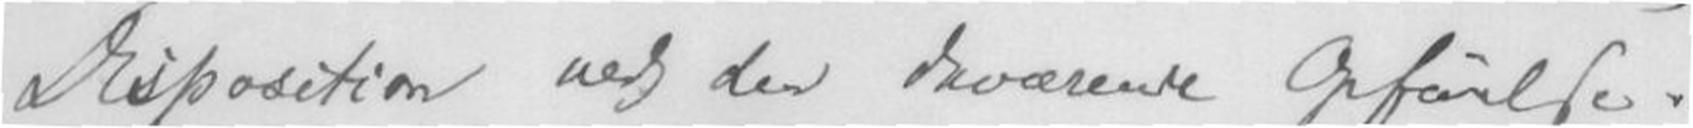Disposition ved den daværende Opførelse.
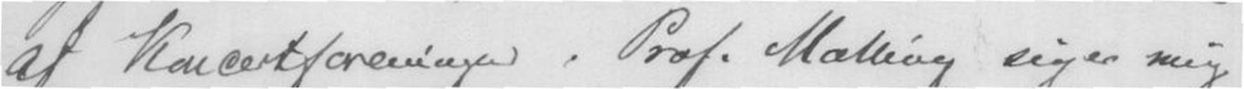af Koncertforeningen. Prof. Malling siger mig
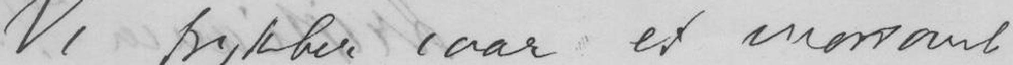Vi trykker iaar et morsomt
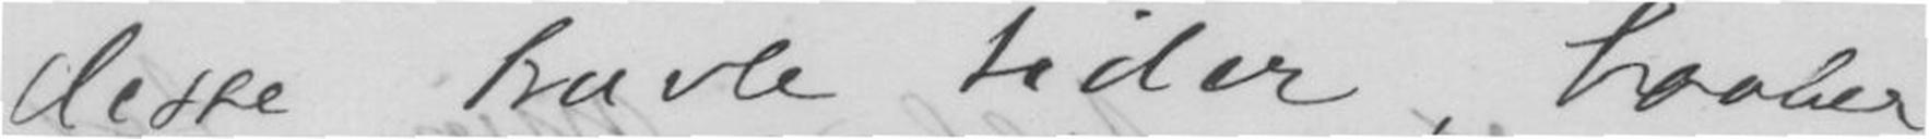disse travle tider, haaber
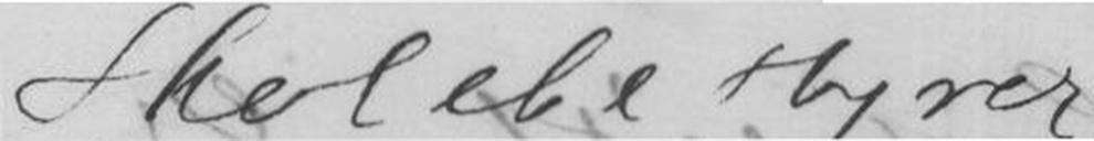Skolebestyrer
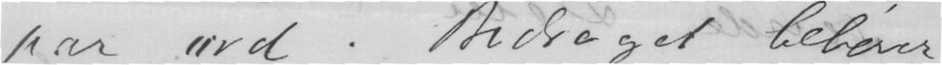par ord. Bidraget behøver
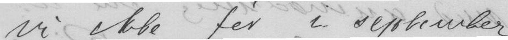vi ikke før i september
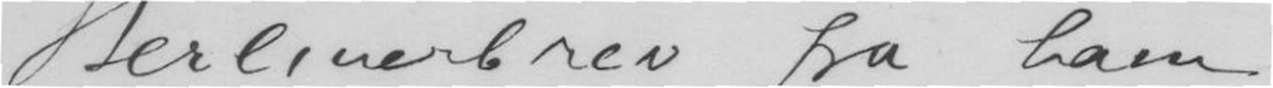Berlinerbrev fra ham
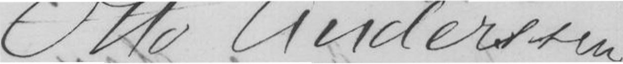Otto Anderssen
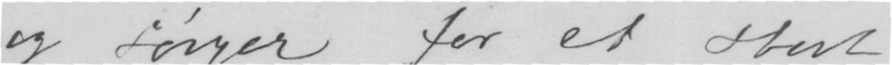og sørger for et stort
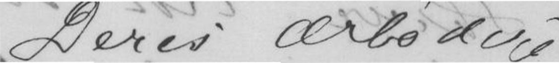Deres Ærbødige
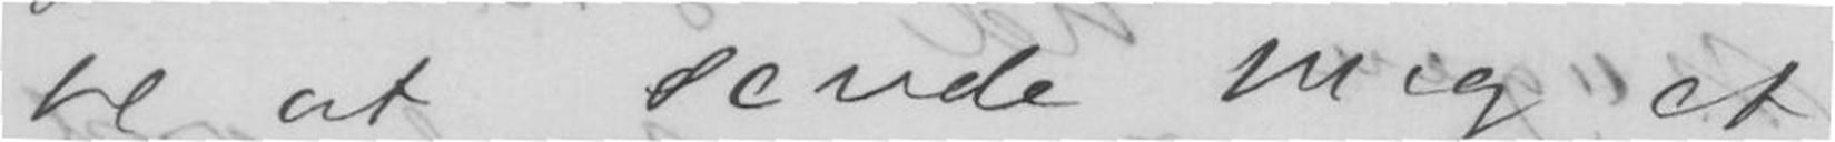at sende mig et
